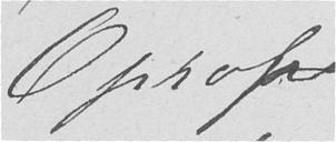Oprop
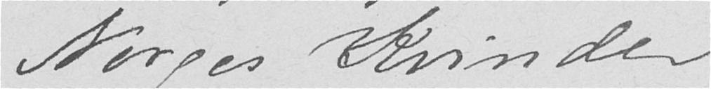Norges Kvinder
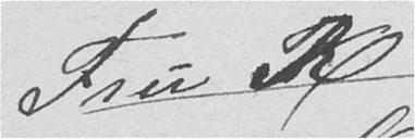Fru R
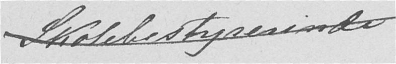Skolebestyrerinde
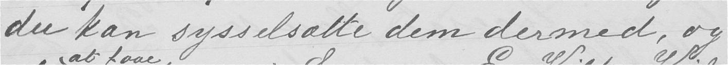du kan sysselsætte dem dermed, og
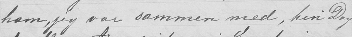ham, jeg var sammen med, hin Dag
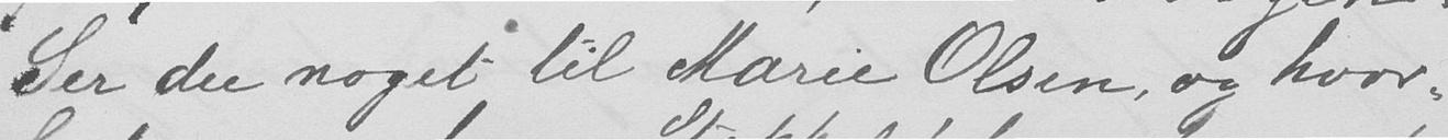Ser du noget til Marie Olsen, og hvor-
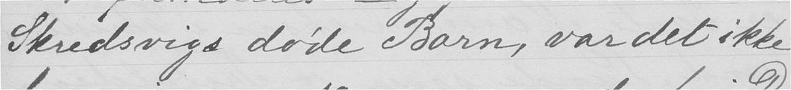Skredsvigs døde Barn, var det ikke
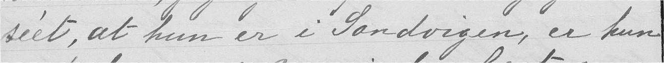séet, at hun er i Sandvigen, er hun
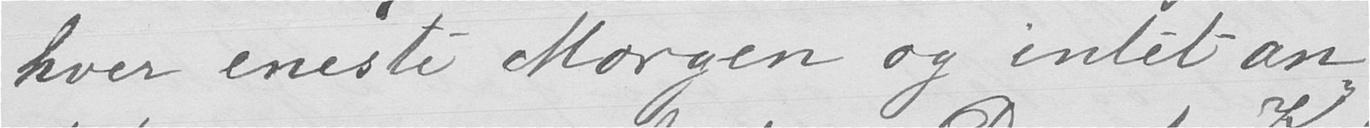hver eneste Morgen og intet an-
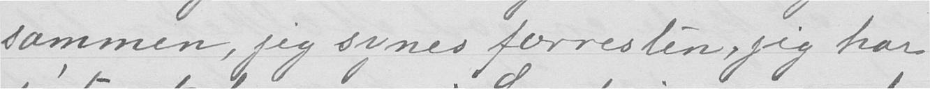sammen, jeg synes forresten, jeg har
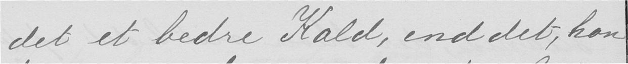det et bedre Kald, end det, han
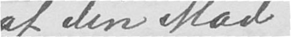af din Mad
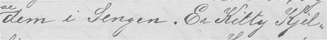dem i Sengen. Er Kitty Kjel-
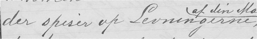der spiser op Levningerne
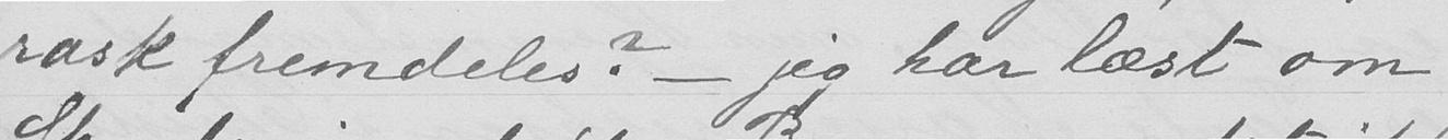rask fremdeles? - jeg har læst om
